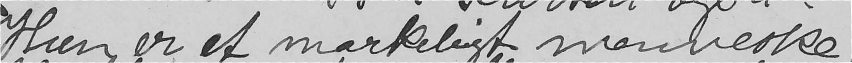Hun er et mærkeligt menneske
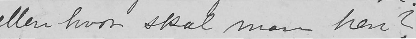Men hvor skal man hen?
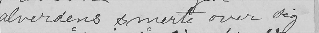alverdens smerte over sig
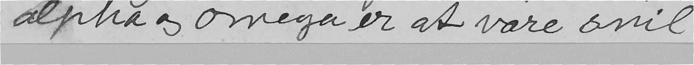alpha og omega er at være snil
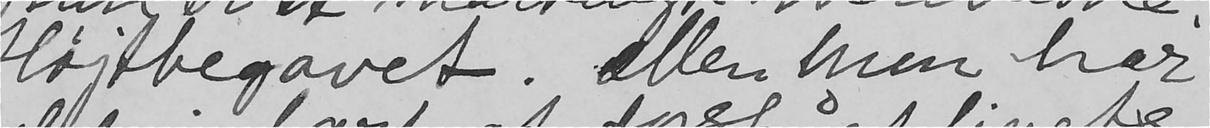Højtbegavet. Men hun har
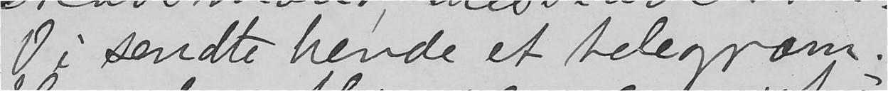Vi sendte hende et telegram.
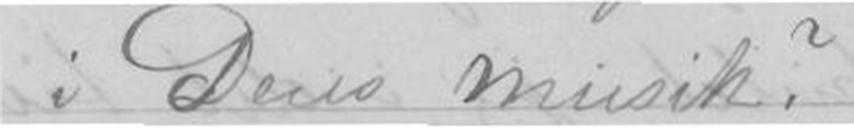i Deres musik?
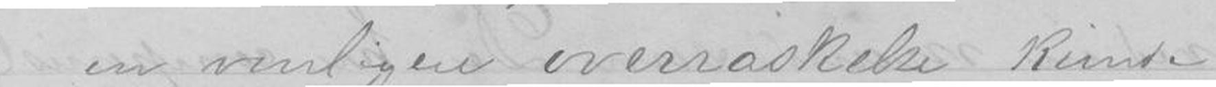en venligere overraskelse kunde
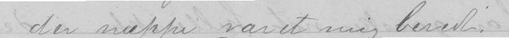der næppe været mig beredt.
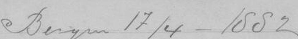Bergen 17/4 - 1882
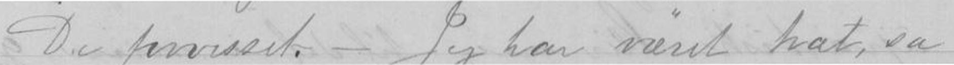De forvisset. - Jeg har väret træt, så
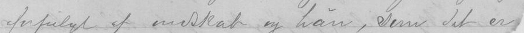forfulgt af ondskab og hån, som det er
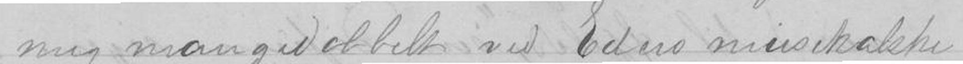mig mangedobbelt ved Eders musikalske
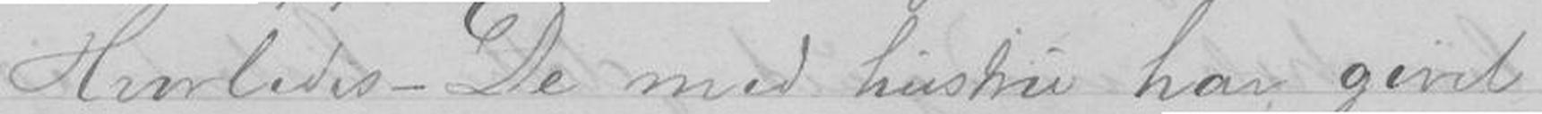Hvorledes - De med hustru har givet
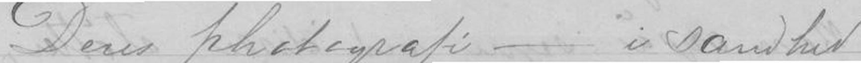Deres Photografi - i sandhed
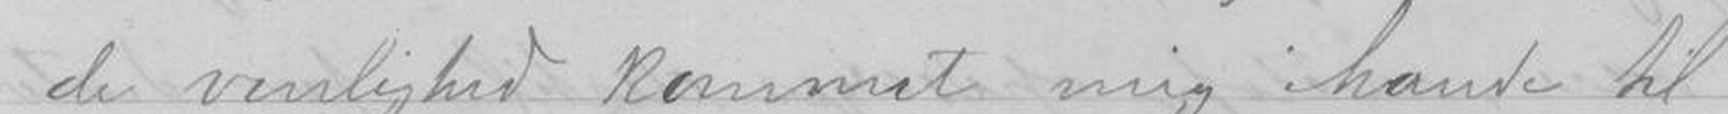de venlighed kommet mig ihænde til
